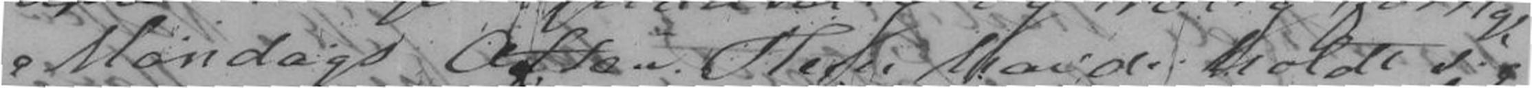Mandags Aften. Hun havde holdt sig
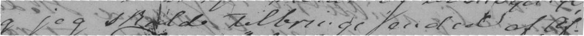og jeg skulde tilbringe endeel af Af-
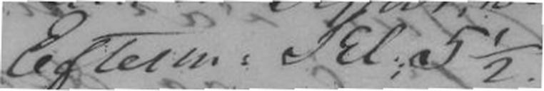Efterm. Kl.5½.
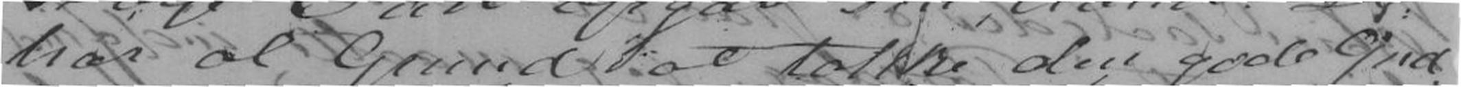har al Grund at takke den gode Gud
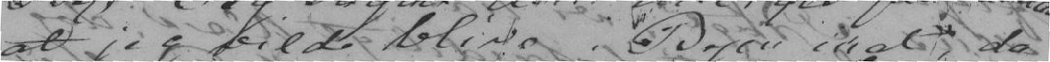at jeg vilde blive i Byen inat, da
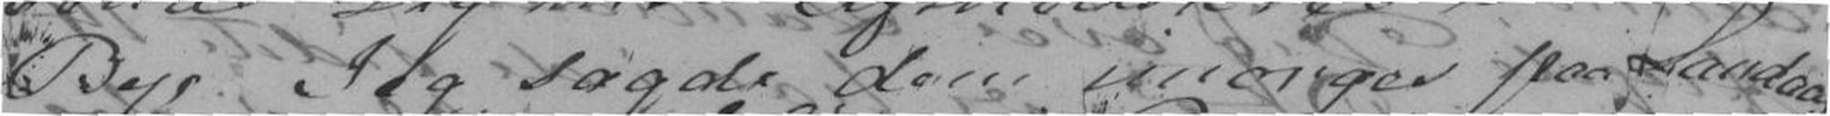By. Jeg sagde dem imorges paa Landaas
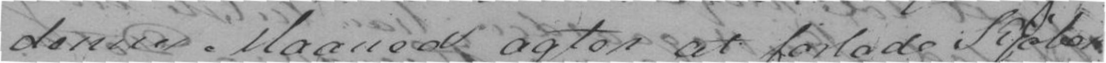denne Maaned agter at forlade Kjøben-
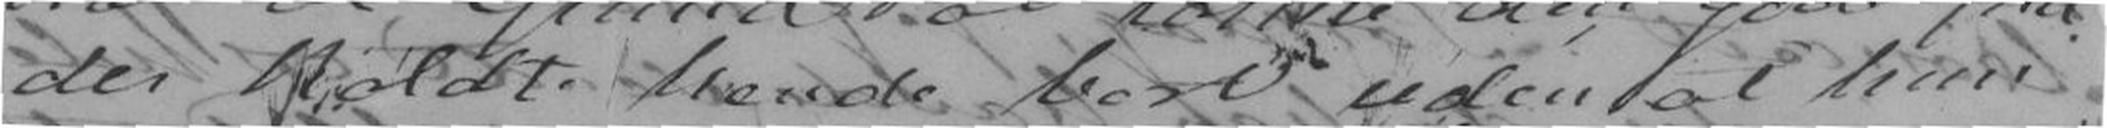der kaldte hende bort uden at hun
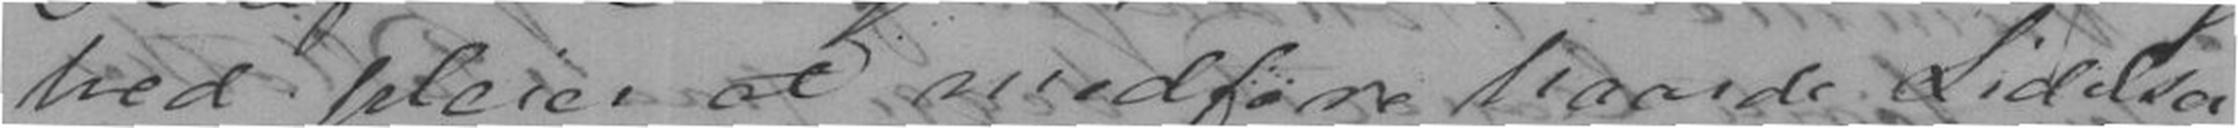hed pleier at medføre haarde Lidelser.
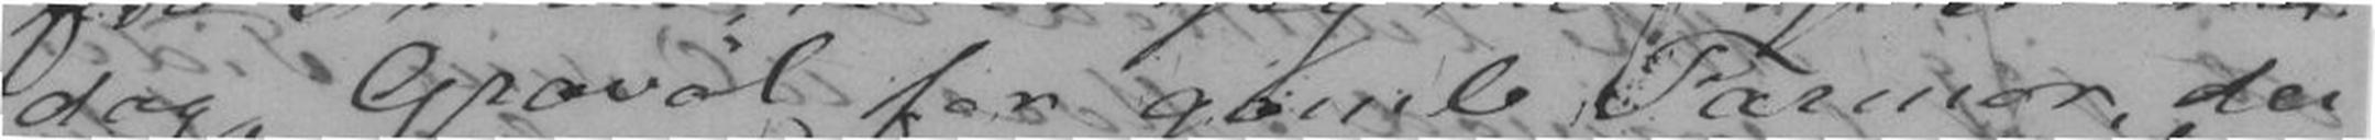dag Gravøl for gamle Farmor, der
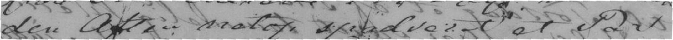den Aften netop spadseret et Par
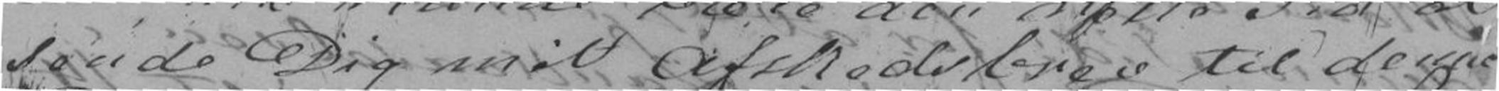sende Dig mit Adskedsbrev til denne
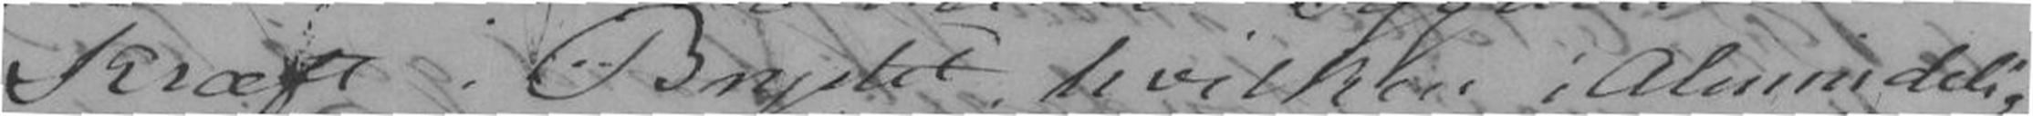Kræft i Brystet hvilken iAlmindelig-
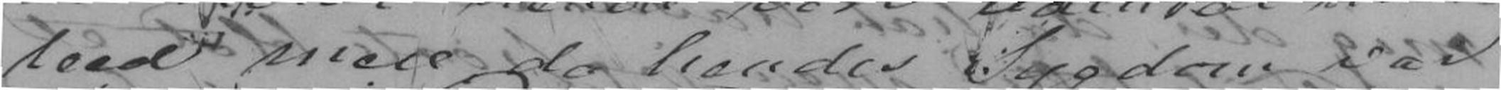leed mere, da hendes Sygdom var
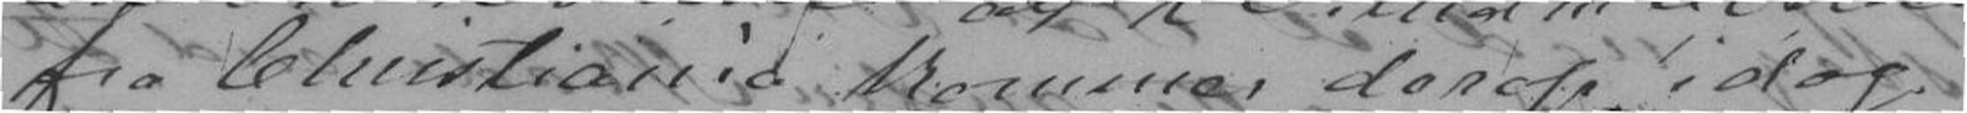fra Christiania kommer derop idag.
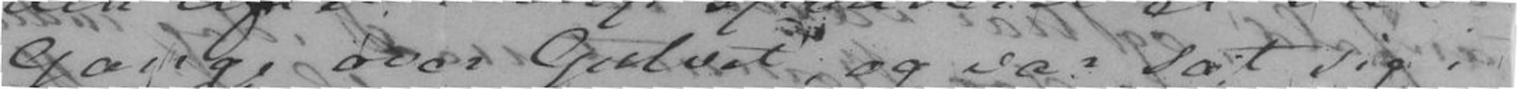Gange over Gulvet, og var sat sig i
